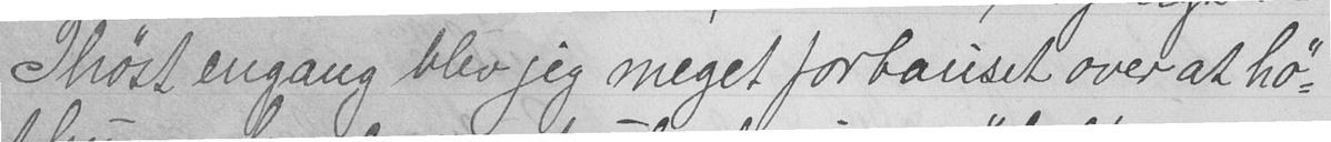Ihøst engang blev jeg meget forbauset over at hø-
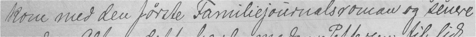kom med den første Familiejournalsroman og senere
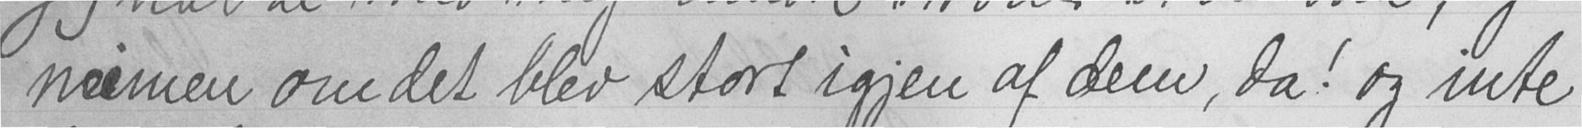næimen om det blev stort igjen af dem, da! og inte
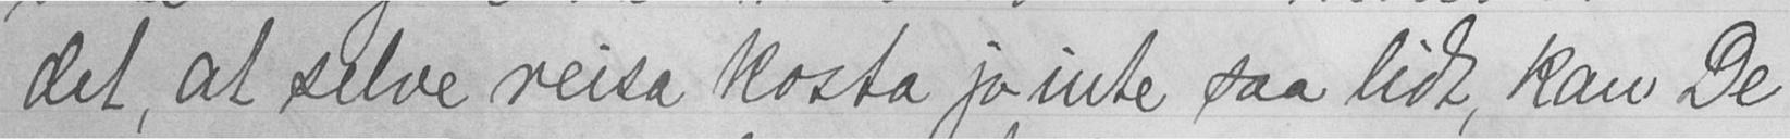det, at selve reisa kosta jo inte saa lidt, kan De
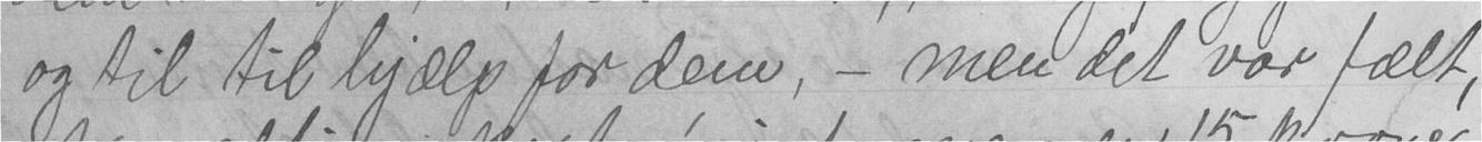og til til hjælp for dem, - men det var fælt,
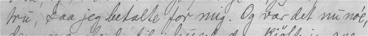tru, saa jeg betalte for mig. Og var det nu noe,
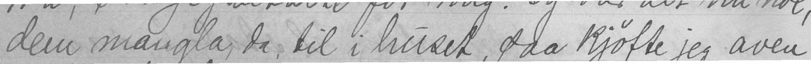dem mangla, da, til i huset, saa kjøfte jeg aven
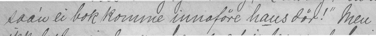saa'n ei bok komme innaføre hans dør!" Men
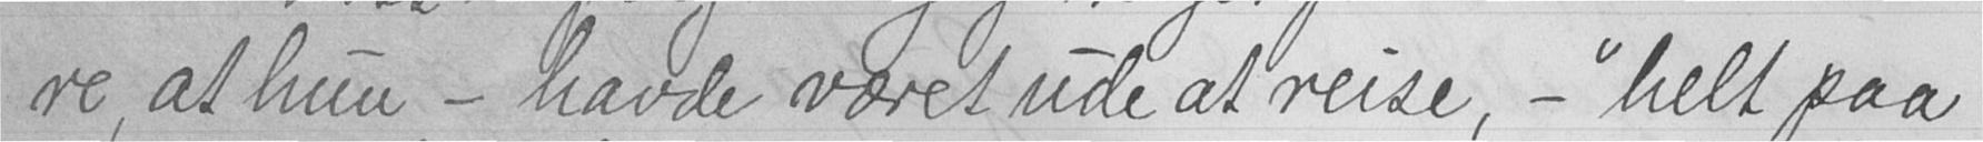re, at hun - havde været ude at reise, - "helt paa
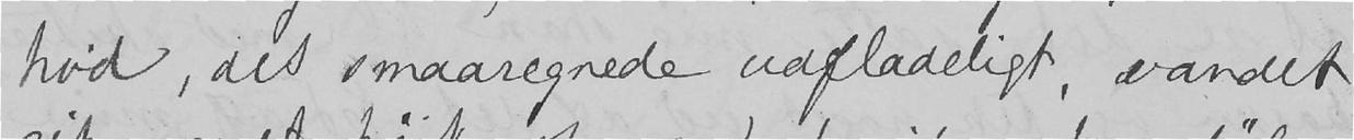hvid, det smaaregnede uafladeligt, vandet
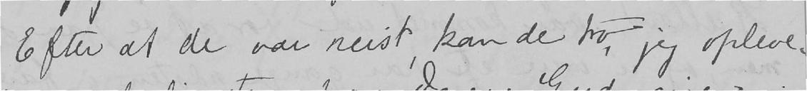Efter at de var reist, kan de tro, jeg oplevede
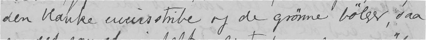den blanke uveirsstribe og de grønne bølger, saa
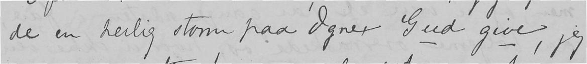en herlig storm paa Ogne, Gud give, jeg
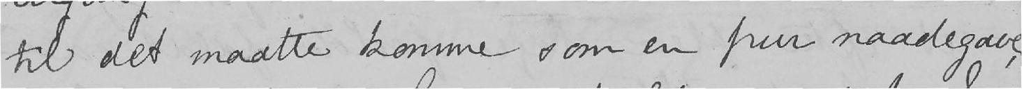til det maatte komme som en pur naadegave,
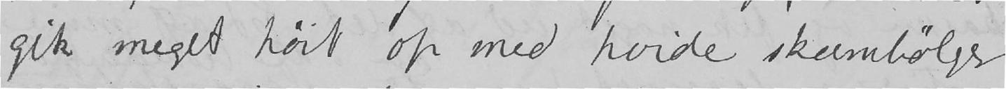gik meget høit op med hvide skumbølger
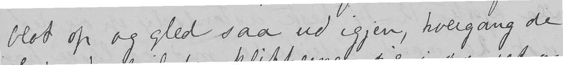blot op og gled saa ud igjen, hvergang de
